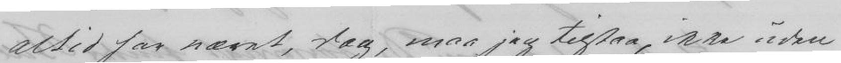altid har næret, dog, maa jeg tilstaa, ikke uden
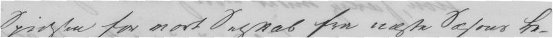i Spidsen for vort Selskab fra næste Sæsons be-
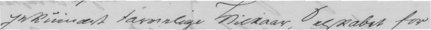pekuniært tarvelige Vilkaar, Selskabet for
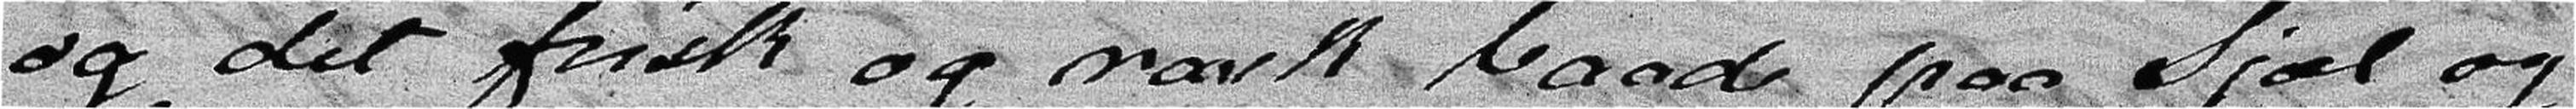og det frisk og rask baade paa Sjæl og
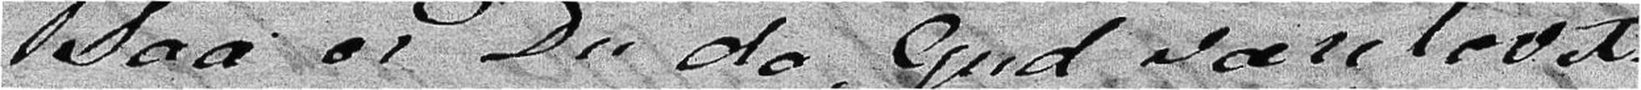Saa er Du da Gud være lovet
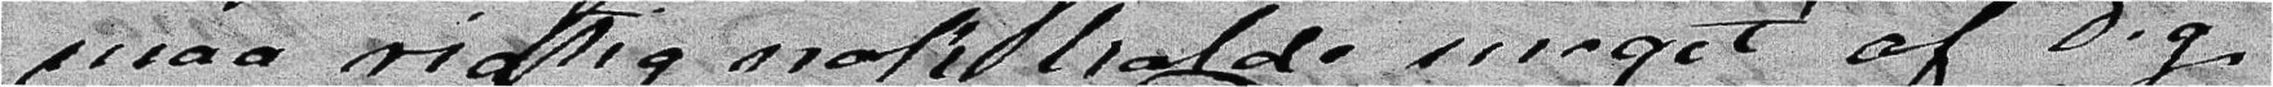maa rigtig nok holde meget af Dig
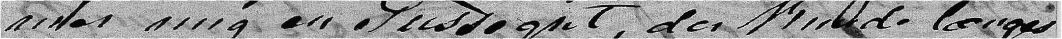mer mig en Tussegut, der kunde længes
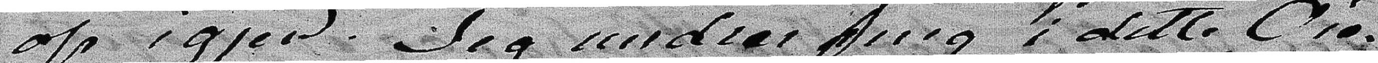op igjen. Jeg undrer mig i dette Øie-
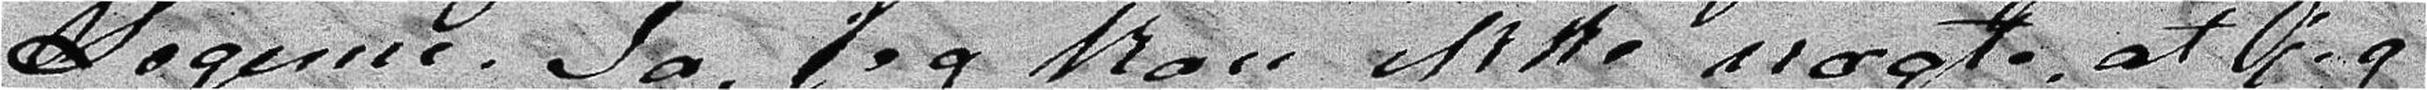Legeme. Ja, jeg kan ikke nægte at jeg
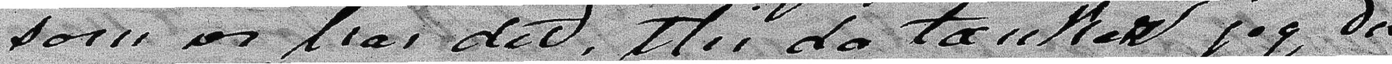som vi har det, thi da tænker jeg der
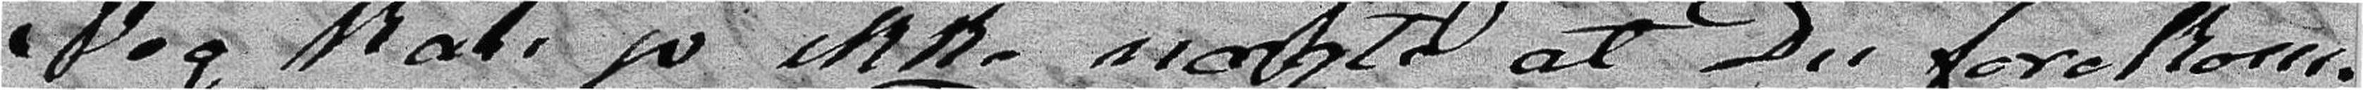Jeg kan jo ikke nægte at Du forekom-
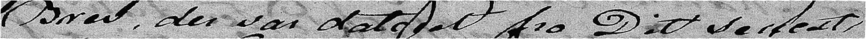Brev, der var dateret fra Dit seneste
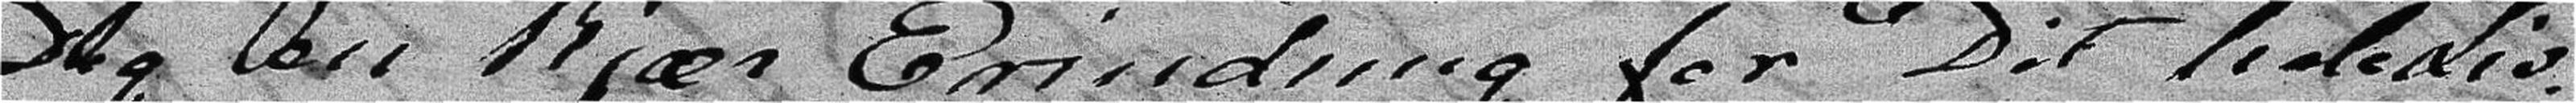Dig en kjær Erindring for Dit hele Liv.
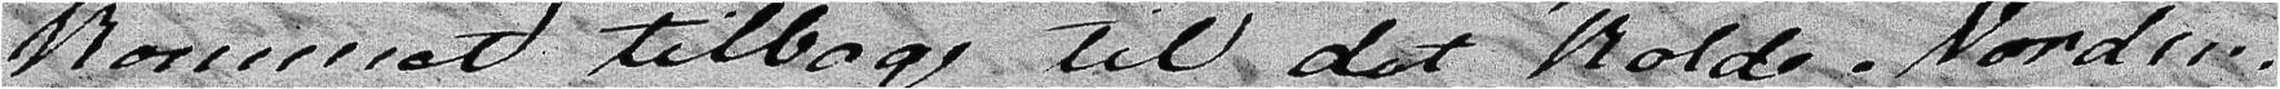kommet tilbage til det kolde Norden,
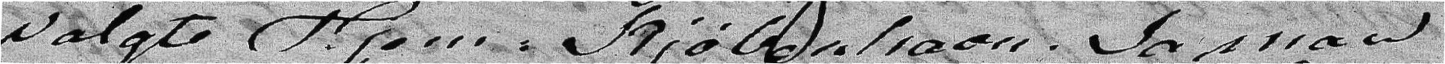valgte Hjem: Kjøbenhavn. Ja man
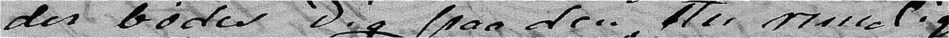der bødes Dig pa den, thi rimelig-
